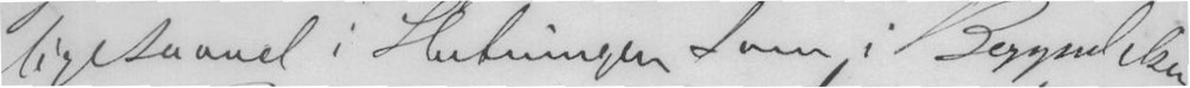ligesaavel i Slutningen som i Begyndelsen
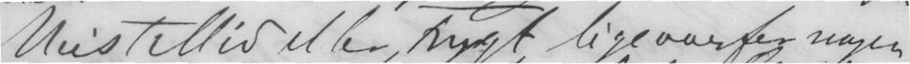Mistillid eller Frygt ligeoverfor nogen
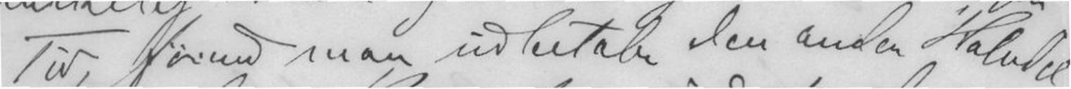Tid, førend man udbetaler den anden Halvdel
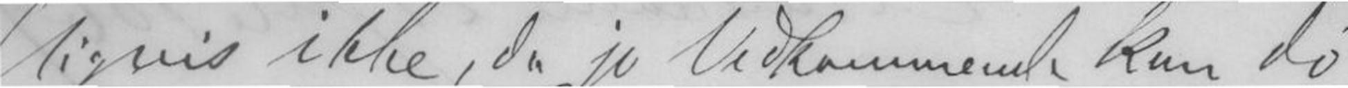ligvis ikke, da jo Vedkommende kan dø
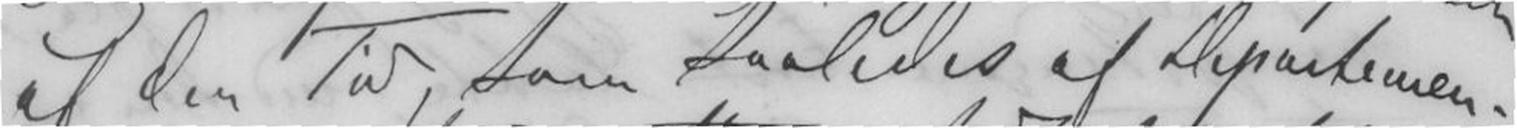af den Tid, som saaledes af Departemen-
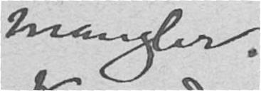mangler.
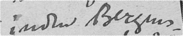inden Bergens-
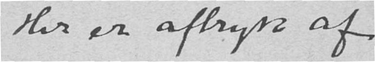Her er aftryk af
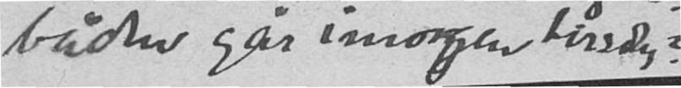båden går imorgen tirsdag?
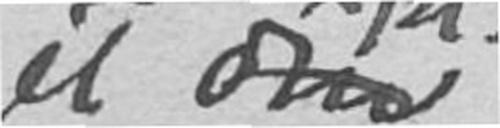et om
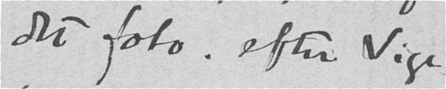det foto. efter Vige-
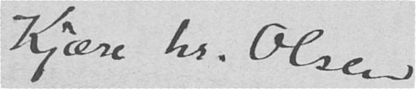Kjære hr. Olsen
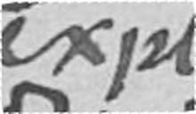expl.
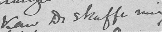Kan De skaffe mig
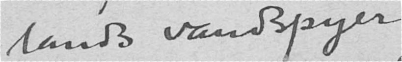lands vandspyer
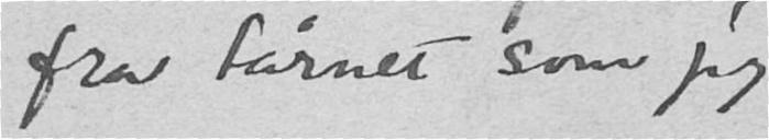fra tårnet som jeg
